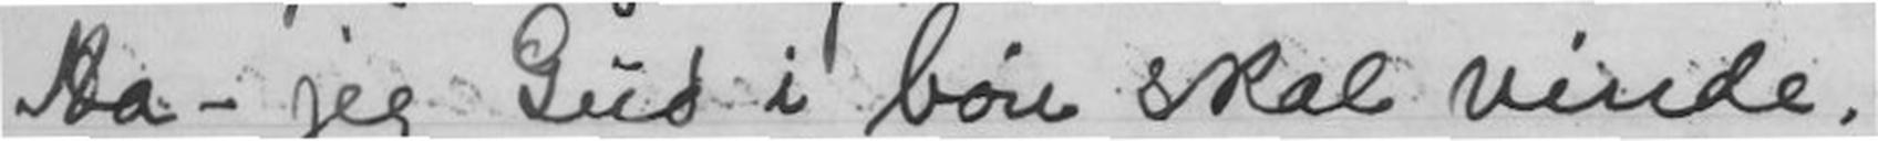Aa - jeg Gud i bøn skal vinde.
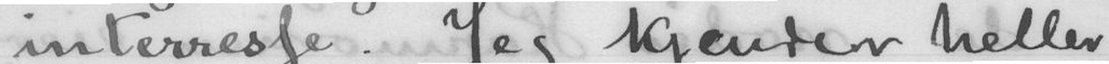interresse. Jeg kjender heller
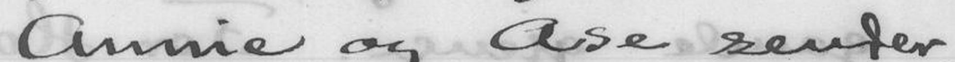Annie og Åse sender
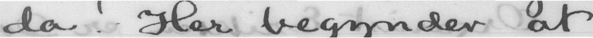da! Her begynder at
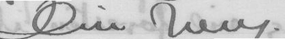Din heng.
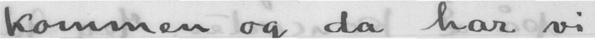kommen og da har vi
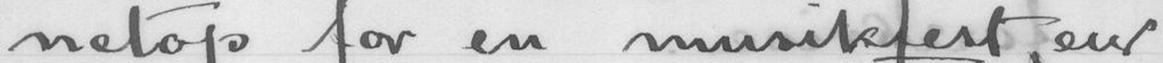netop for en musikfest, end
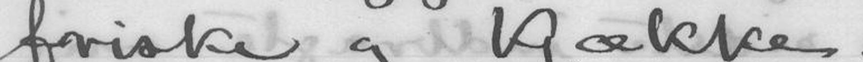friske og kjække.
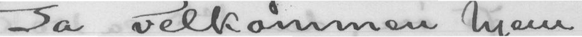Ja, velkommen hjem
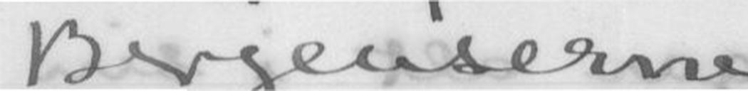Bergenserne
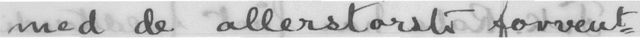med de allerstørste forvent-
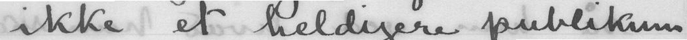ikke et heldigere publikum
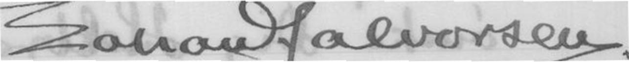Johan Halvorsen
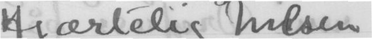Hjærtelig hilsen
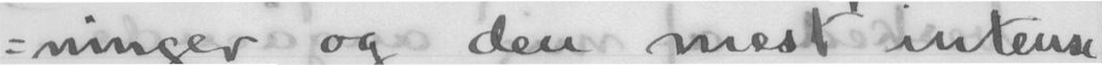ninger og den mest intense
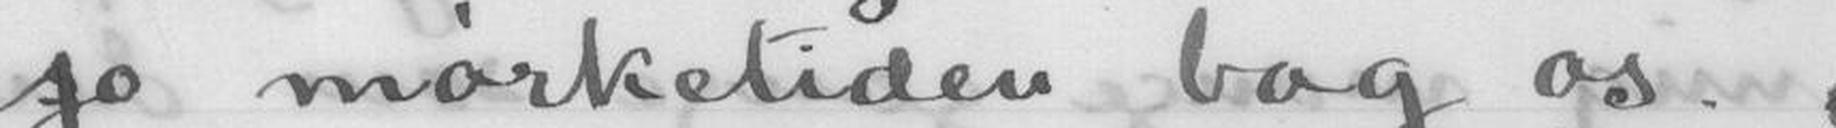jo mørketiden bag os.
